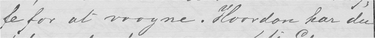fe for at vaagne. Hvordan har du
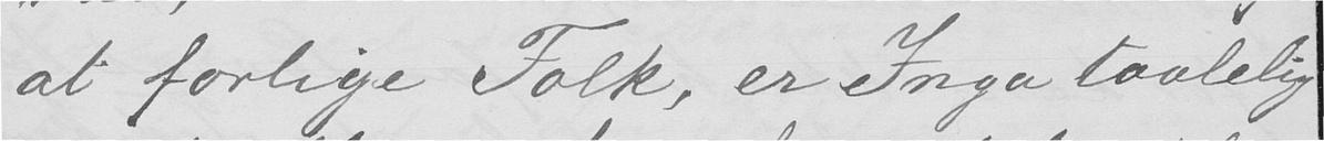at forlige Folk, er Inga taalelig
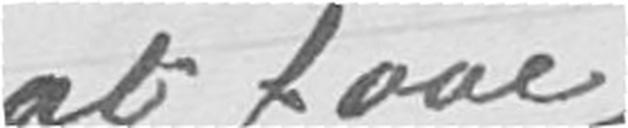at faae
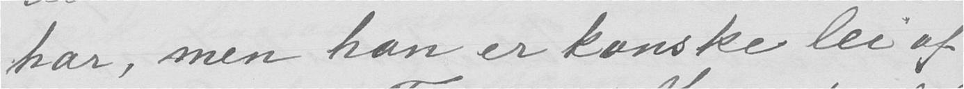har, men han er kanske lei af
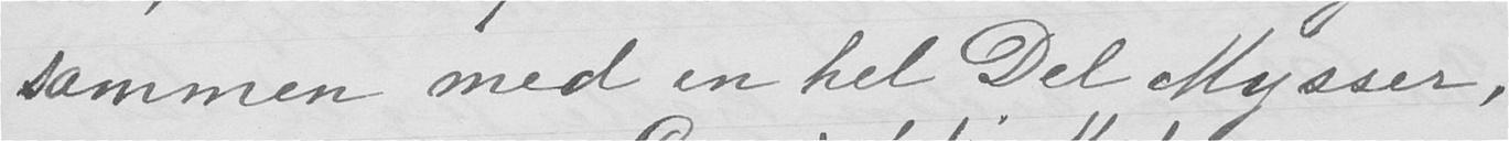sammen med en hel Del Mysser,
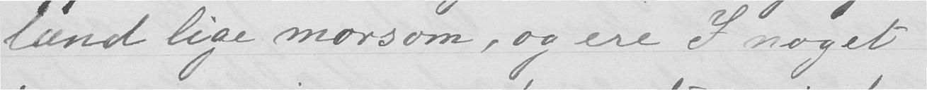land lige morsom, og ere I noget
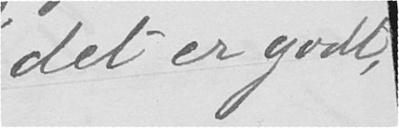det er godt,
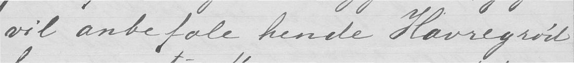vil anbefale hende Havregrød
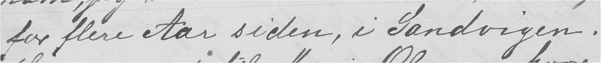for flere Aar siden, i Sandvigen.
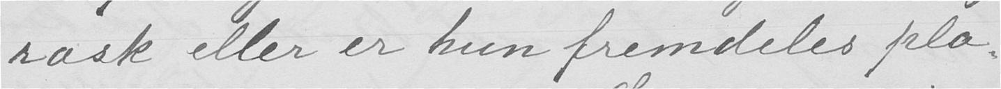rask eller er hun fremdeles pla-
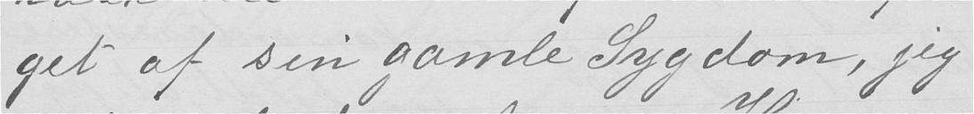get af sin gamle Sygdom, jeg
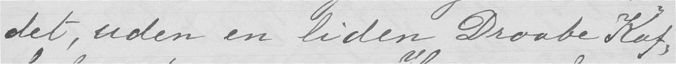det, uden en liden Draabe Kaf-
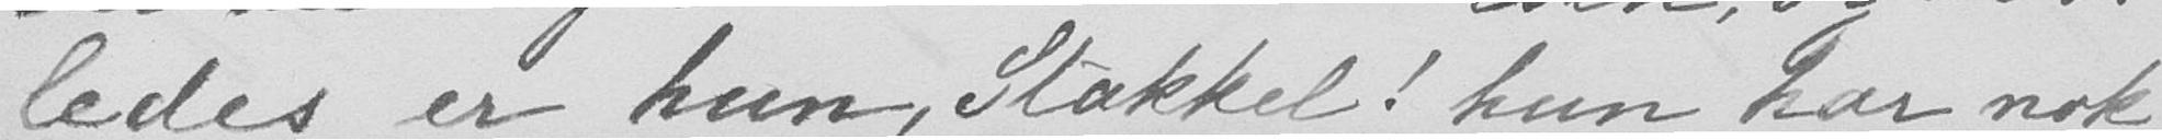ledes er hun, Stakkel! hun har nok
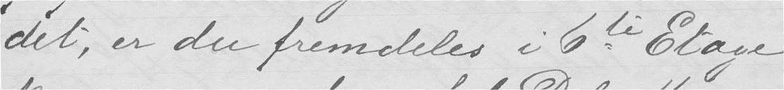det, er du fremdeles i 6te Etage
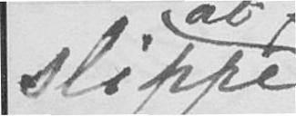slippe
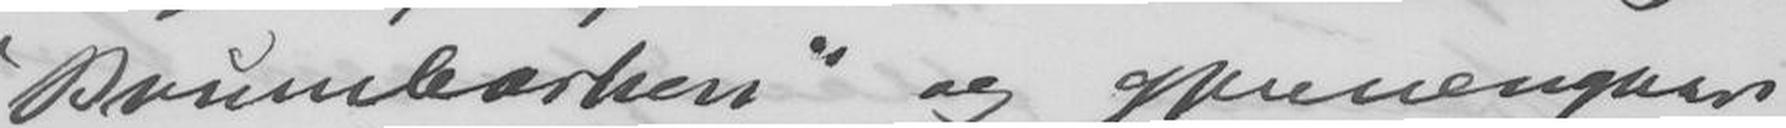"Brumbarken" og gjennemgaar
